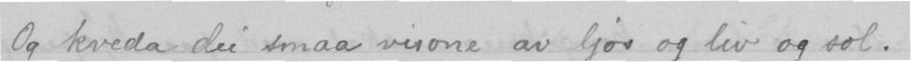Og kveda dei smaa visone av ljos og liv og sol.
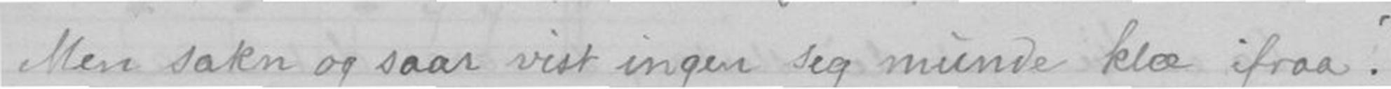Men sakn og saar vist ingen seg munde klæ ifraa.
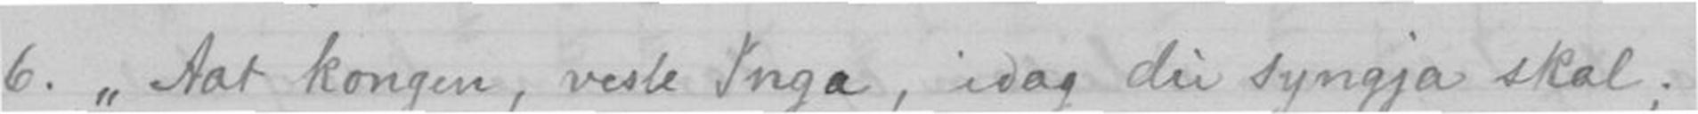6. Aat kongen, vesle Inga, idag du syngja skal;
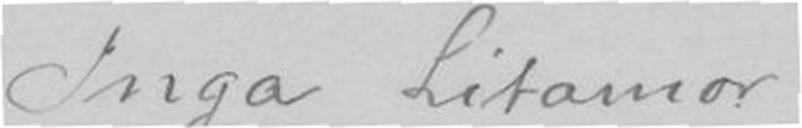Inga Litamor
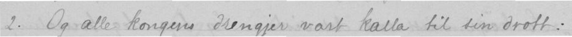2. Og alle kongens drengjer vart kalla til sin drott:
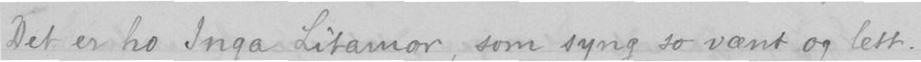Det er ho Inga Litamor, som syng so vænt og lett.
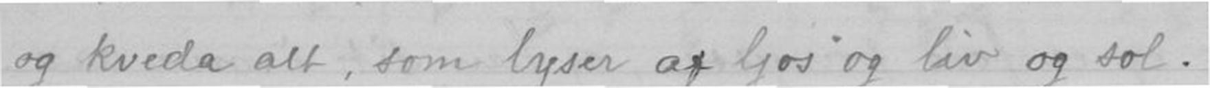og kveda att, som lyser af ljos og liv og sol.
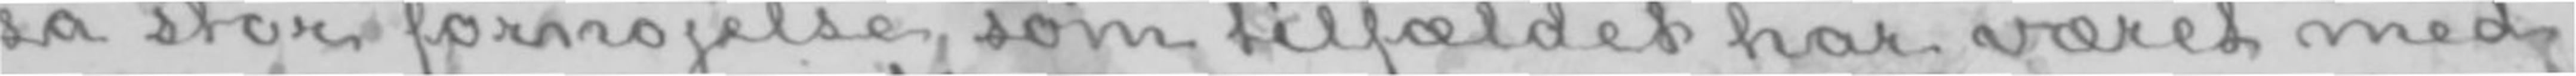så stor fornøjelse som tilfældet har været med
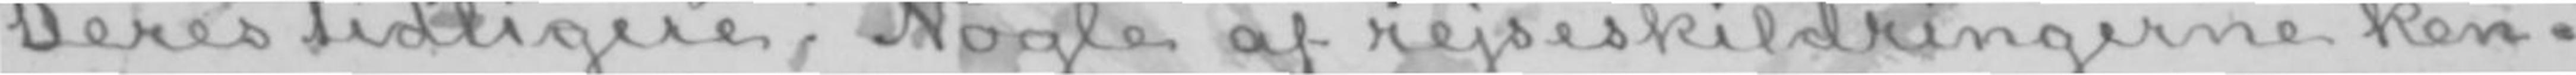Deres tidligere. Nogle af rejseskildringerne ken-
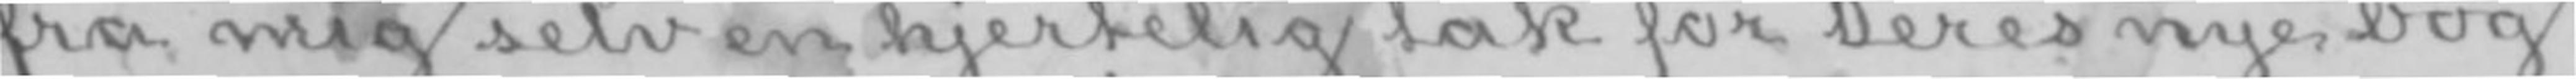fra mig selv en hjertelig tak for Deres nye bog,
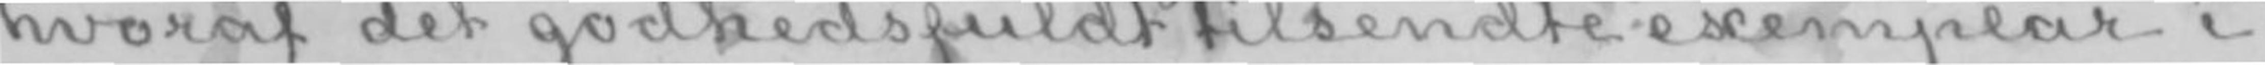hvoraf det godhedsfuldt tilsendte exemplar i-
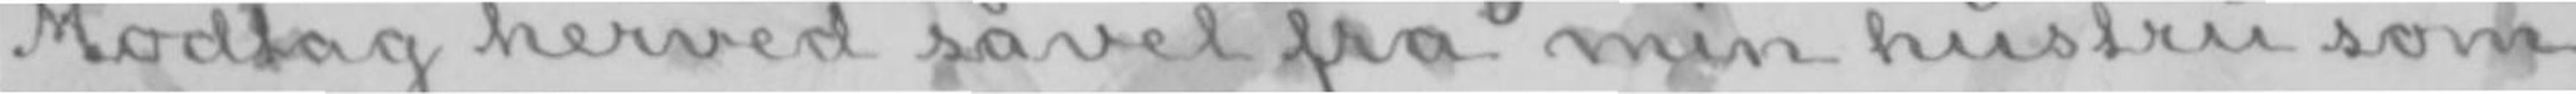Modtag herved såvel fra min hustru som
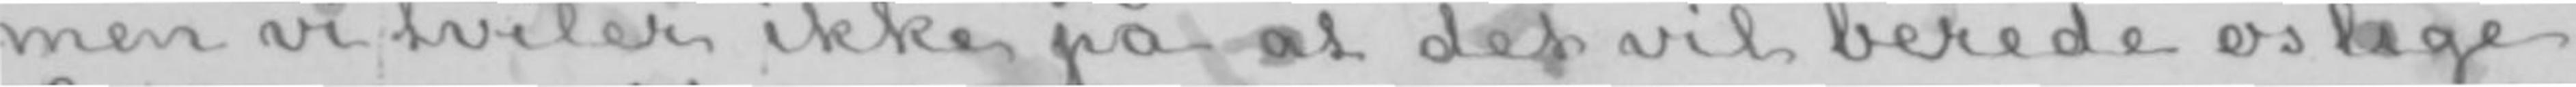men vi tviler ikke på at det vil berede os lige
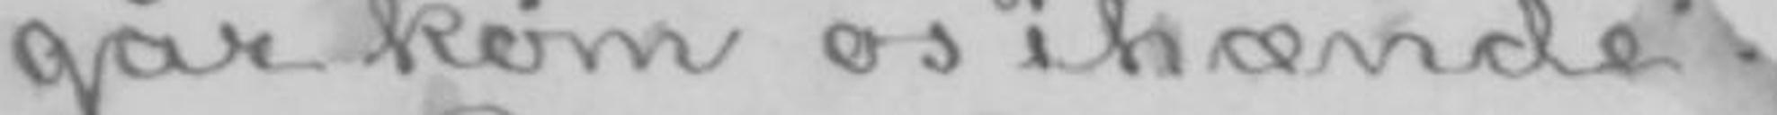går kom os ihænde.
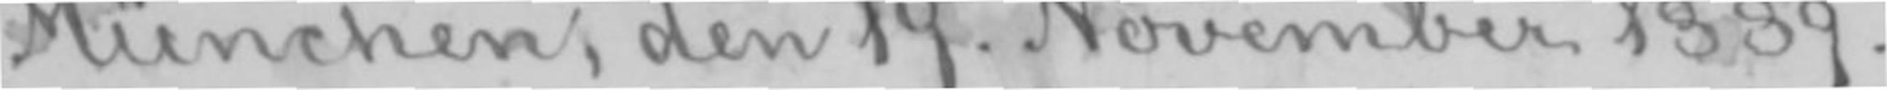München, den 19. November 1889.
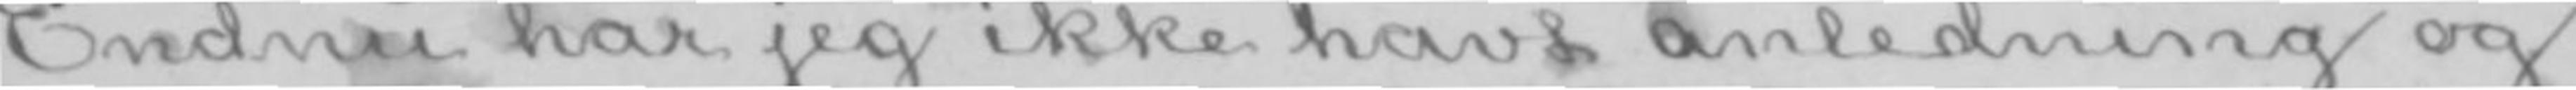Endnu har jeg ikke havt anledning og
